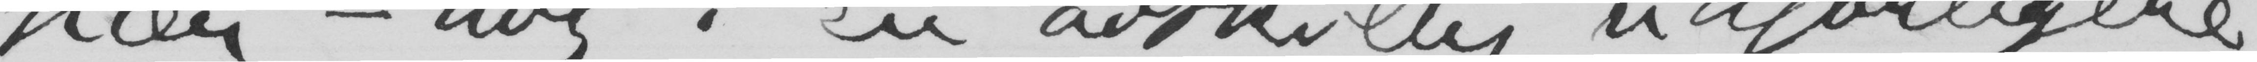hær – dog i en adskillig udførligere
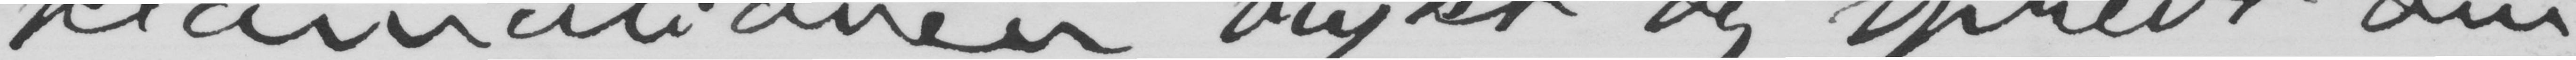klamationen trykt og spredt om-
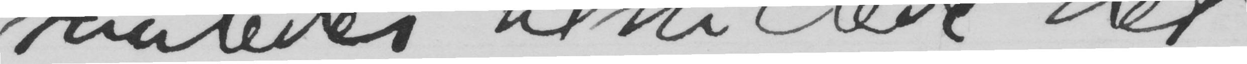saaledes tilstillede det.
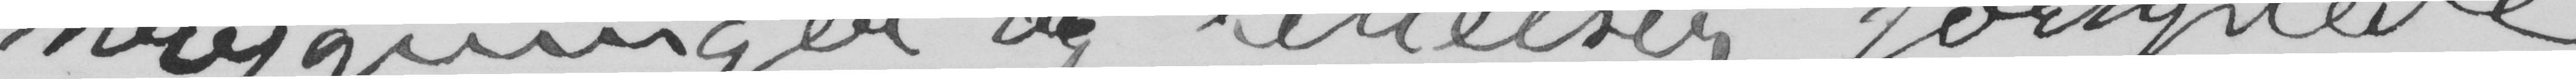strygninger og rettelser forsynede
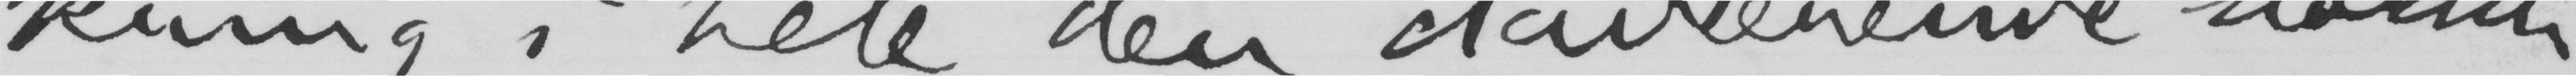kring i hele den daværende norske
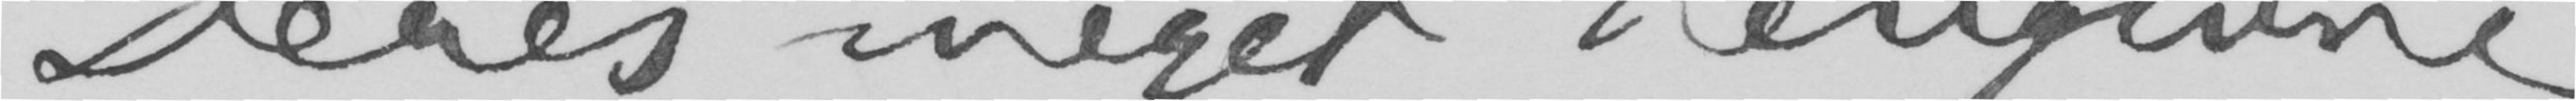Deres meget hengivne
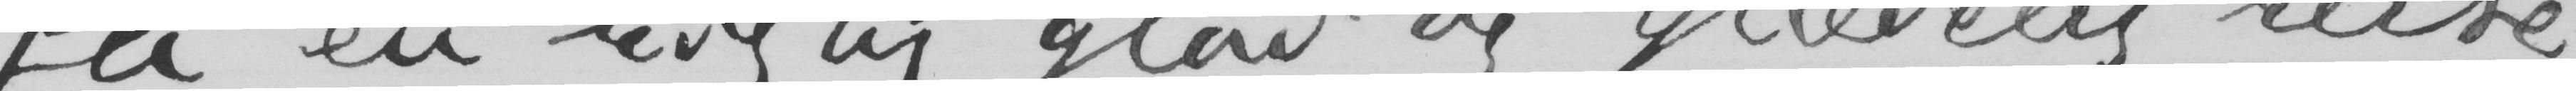Ja, en rigtig glad og glædelig reise
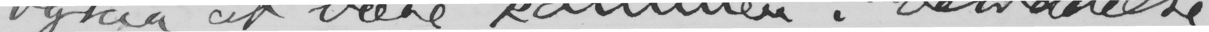ogsaa at være kommen i besiddelse
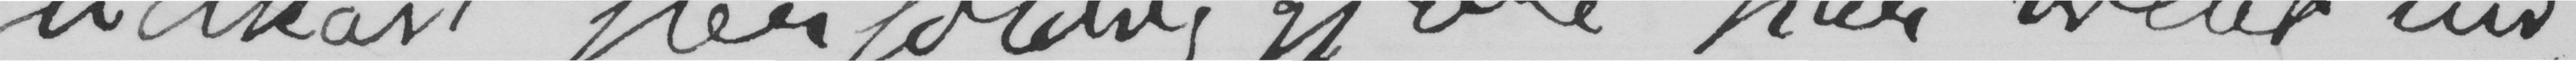udkast flerfoldiggjøre har villet ud-
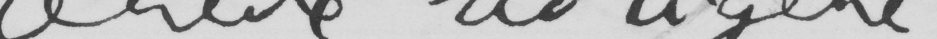lerede tidligere
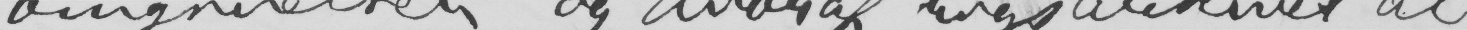omgivelser, og hvoraf rigsarkivet al-
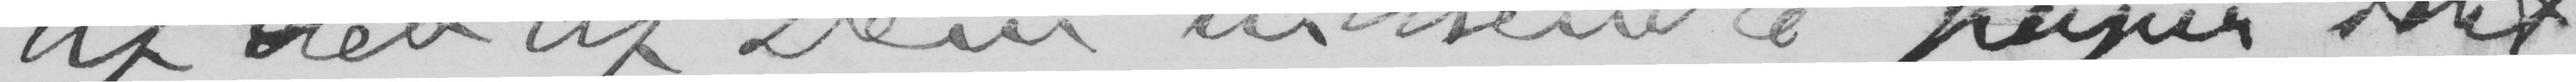af det af Dem indsendte papir, idet
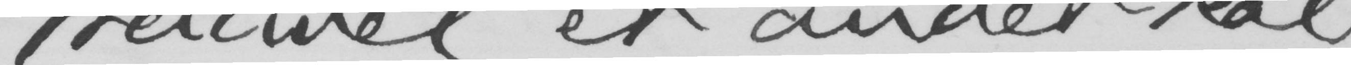saavel et andet kal-
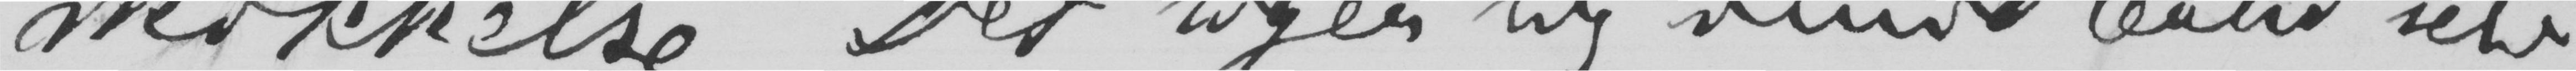skikkelse. Det siger sig imidlertid selv,
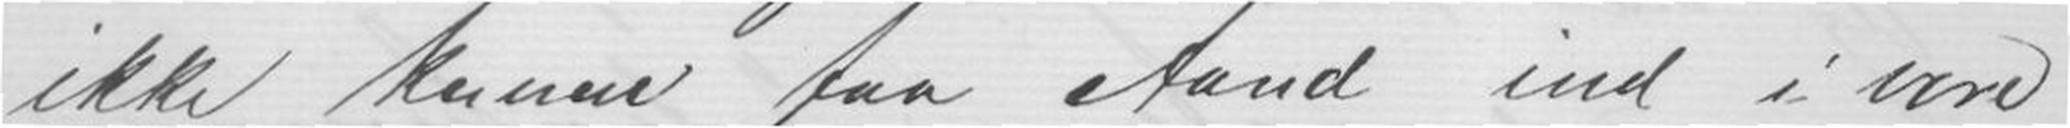ikke kunne faa Aand ind i vore
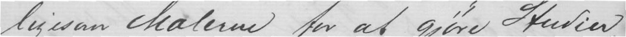ligesom Malerne for at gjøre Studier.
